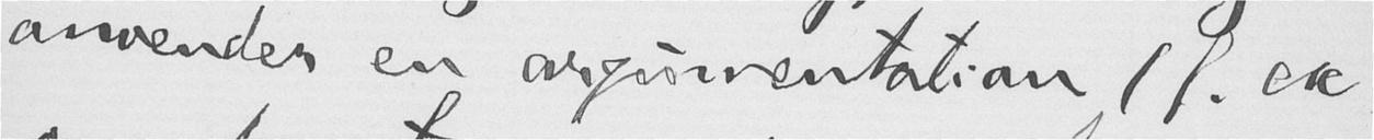anvender en argumentation (f. ex.
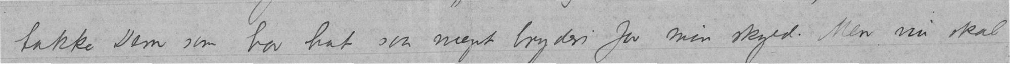takke Dem som har hat saa meget bryderi for min skyld. Men nu skal
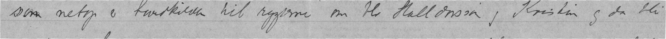som netop er hovedkilden til rygterne om Ulv Halldorsson og Kristin og da blir
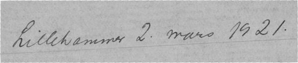Lillehammer 2. mars 1921.
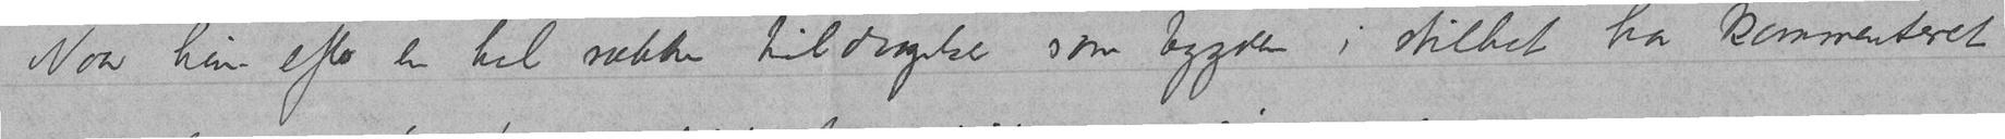Naar hun efter en hel række tildragelse som bygden i stilhet har kommenteret
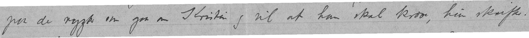paa de rygter som gaar om Kristin og vil at han skal kræve, hun skrifter.
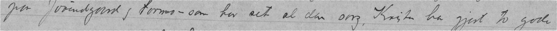paa Jørundgaard og Formo – som har set al den sorg Kristin har gjort to gode
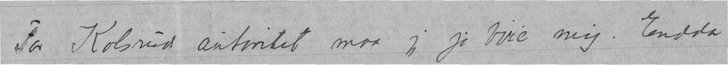For Kolsruds autoritet maa jeg jo bøie mig. Endda
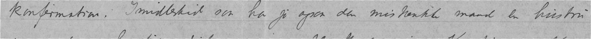konfirmation. Imidlertid saa har jo ogsaa den mistænkte mand en hustru
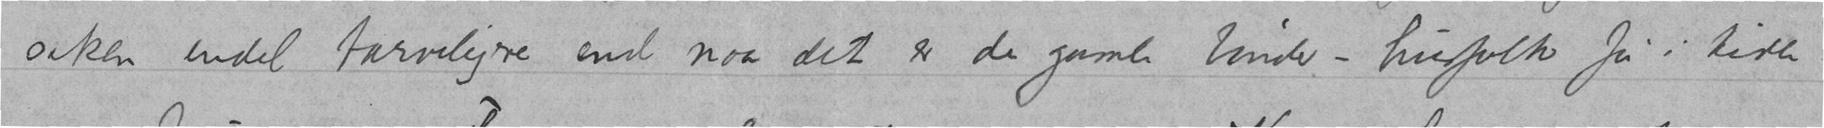saken en del tarveligere end naar det er de gamle bønder – husfolk før i tiden,
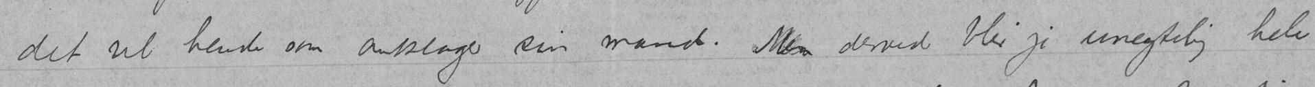det vel hende som anklager sin mand. Men derved blir jo unegtelig hele
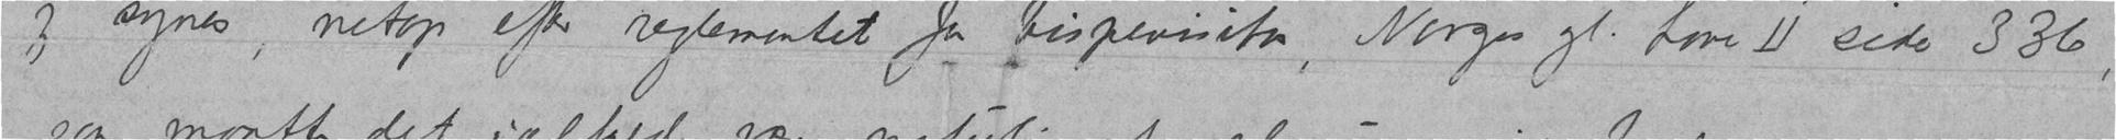jeg synes, netop efter reglementet for bispevisitas, Norges gl. Love II side 336,
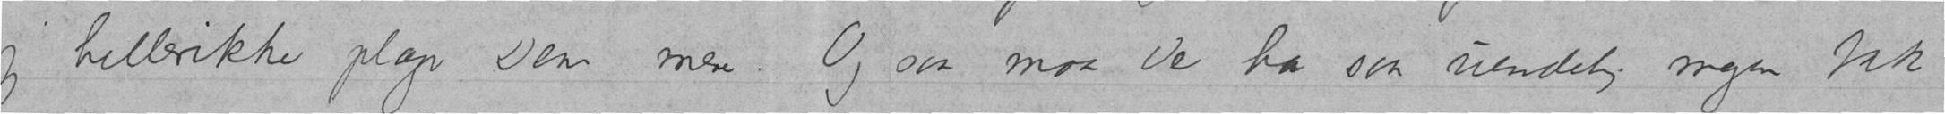jeg hellerikke plage Dem mere. Og saa maa De ha saa uendelig megen tak
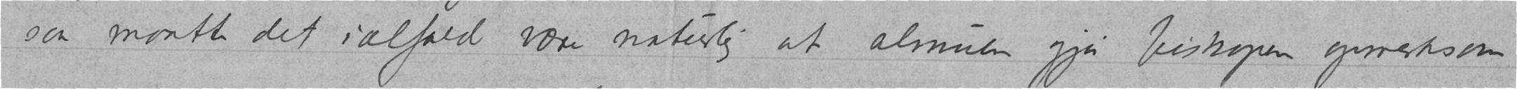saa maatte det ialfald være naturlig at almuen gjør biskopen opmerksom
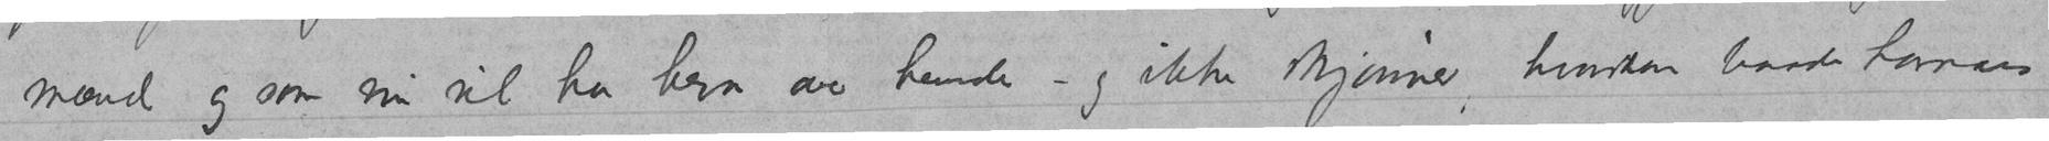mænd og som nu vil ha hevn over hende – og ikke skjønner, hvordan baade Lavrans
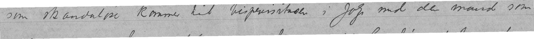som skandaløse kommer til bispevisitasen i følge med den mand som
